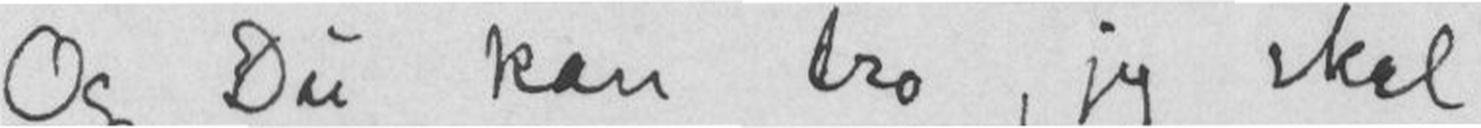Og Du kan tro, jeg skal
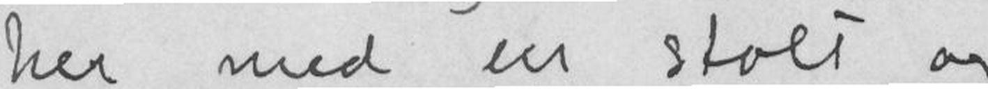her med en stolt og
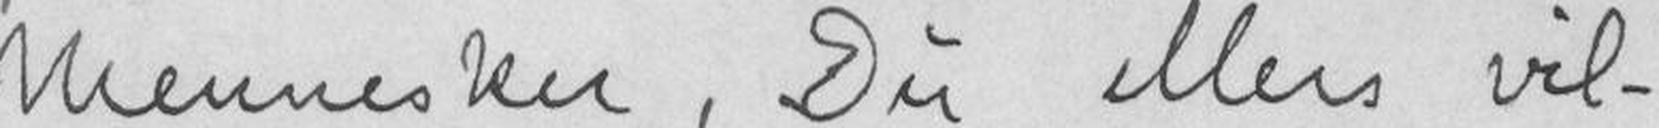Mennesker, Du ellers vil-
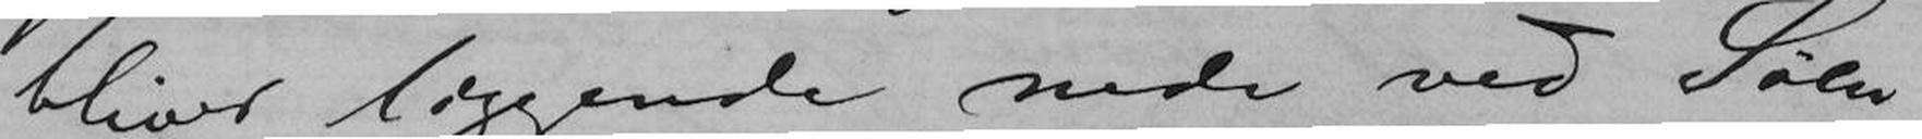bliver liggende nede ved Søen.
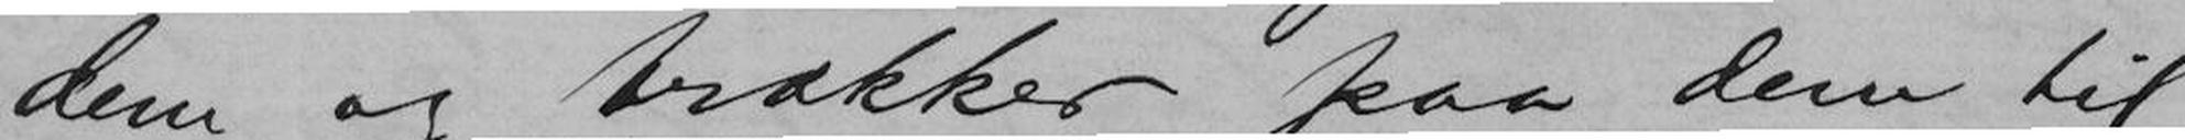dem og trakker paa dem til
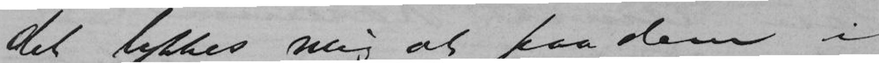det lykkes mig at faa dem i
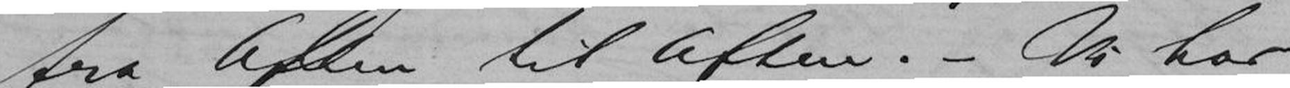fra Aften til Aften. - Vi har
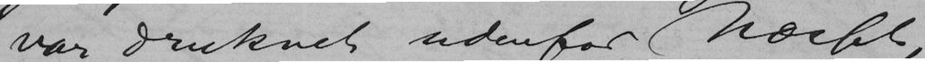var druknet udenfor Næsset,
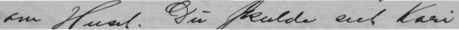om Huset. Du skulde seet Kari
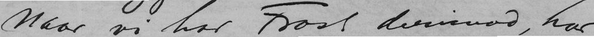Naar vi har Frost derimod, har
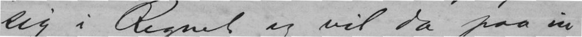sig i Regnet og vil da paa in-
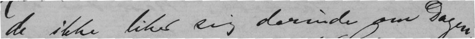de ikke liker sig derinde om Dagen.
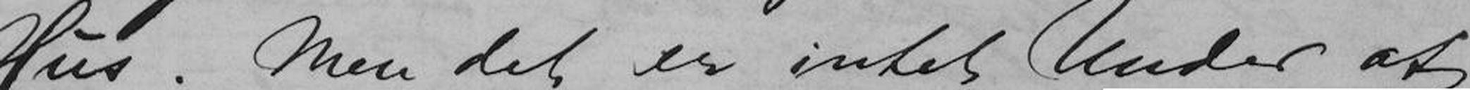Hus. Men det er intet Under at
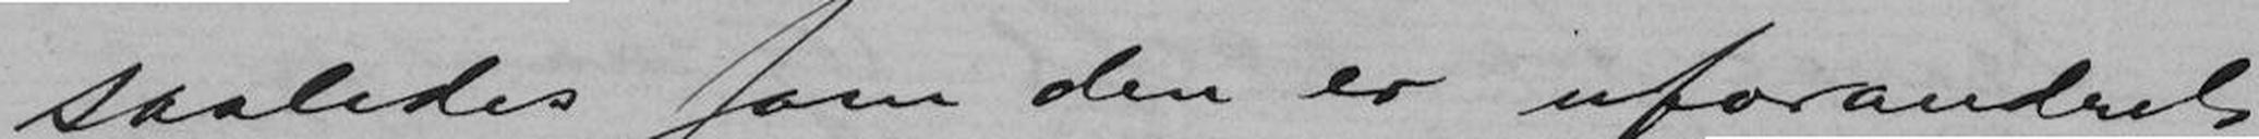saaledes som den er uforandret
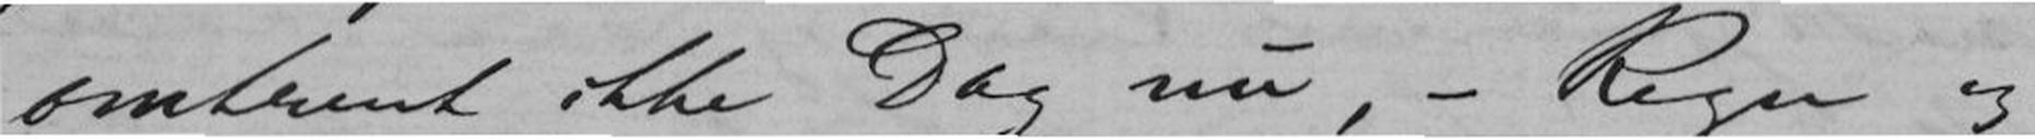omtrent ikke Dag nu, - Regn og
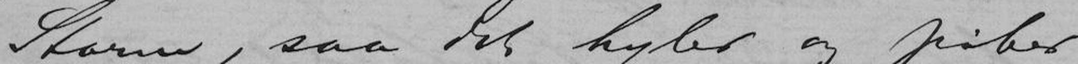Storm, saa det hyler og piber
